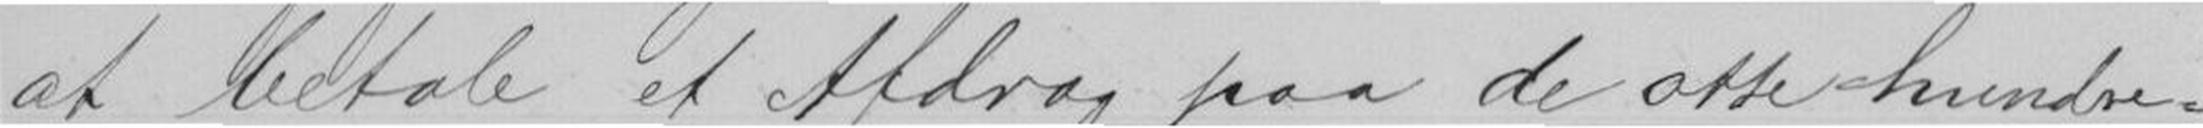at betale et Afdrag paa de ottehundre-
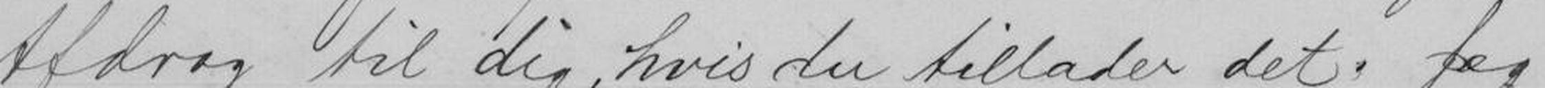Afdrag til dig, hvis du tillader det. Jeg
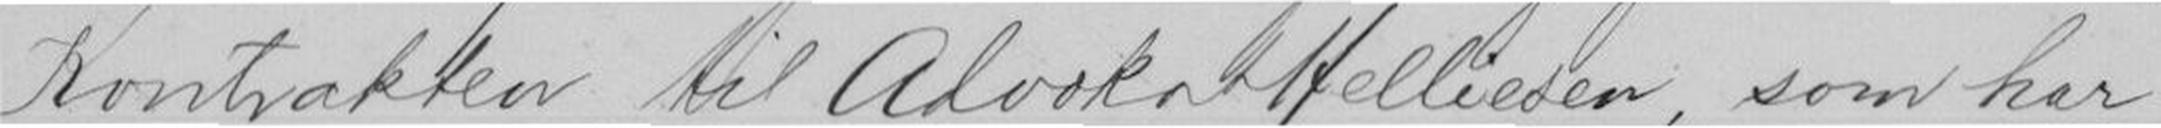Kontrakten til Advokat Helliesesn, som har
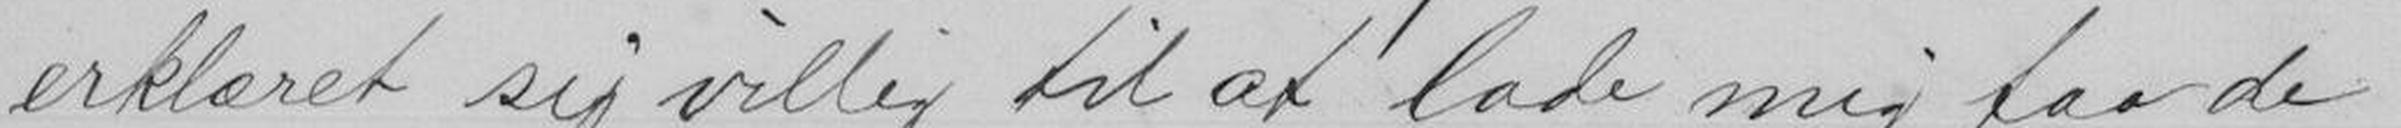erklært sig villig til at lade mig faa de
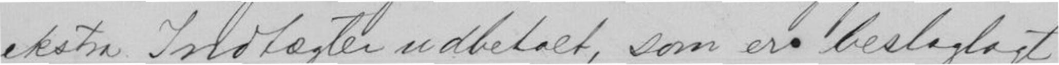ekstra Indtægter udbetalt, som er beslaglagt
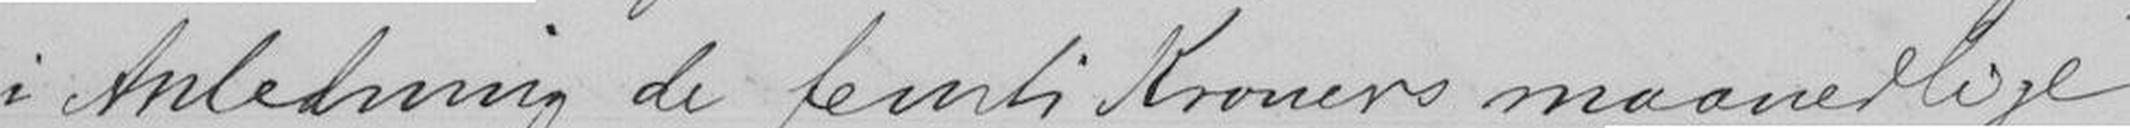i Anledning de femti Kroners maandeslige
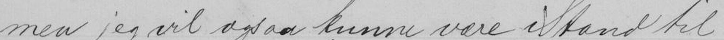men jeg vil ogsaa kunne være istand til
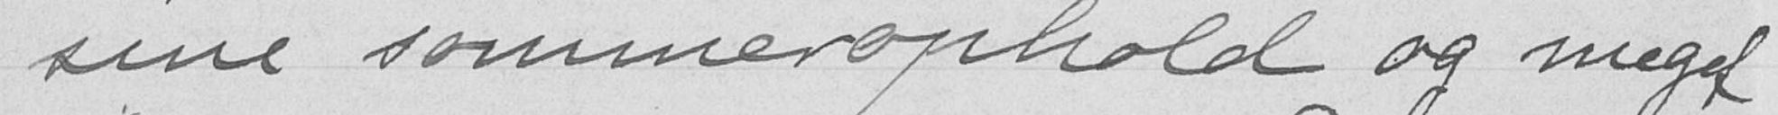sine sommerophold og meget
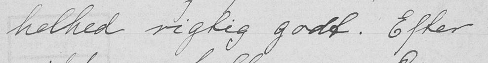helhed rigtig godt. Efter
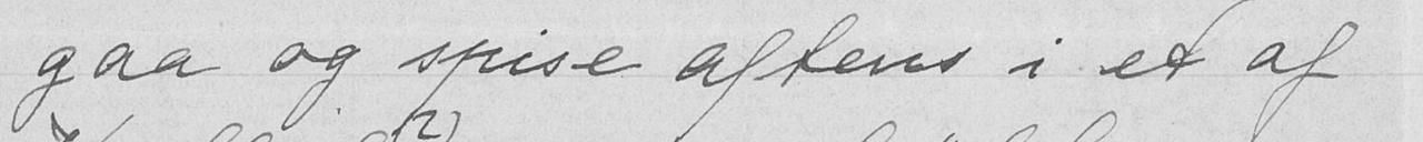gaa og spise aftens i et af
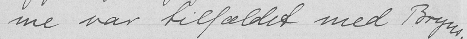me var tilfældet med Bryns,
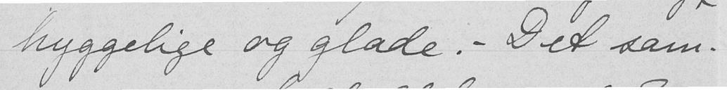hyggelige og glade.- Det sam-
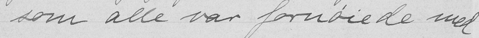som alle var fornøiede med
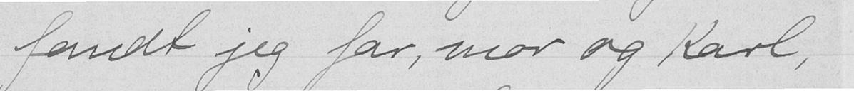fandt jeg far, mor og Karl,
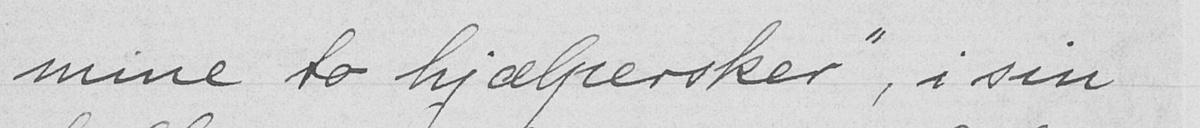mine to hjælpersker, i sin
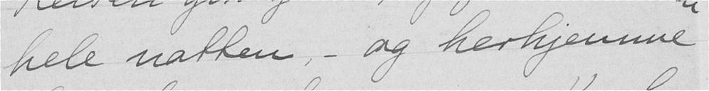hele natten, - og herhjemme
